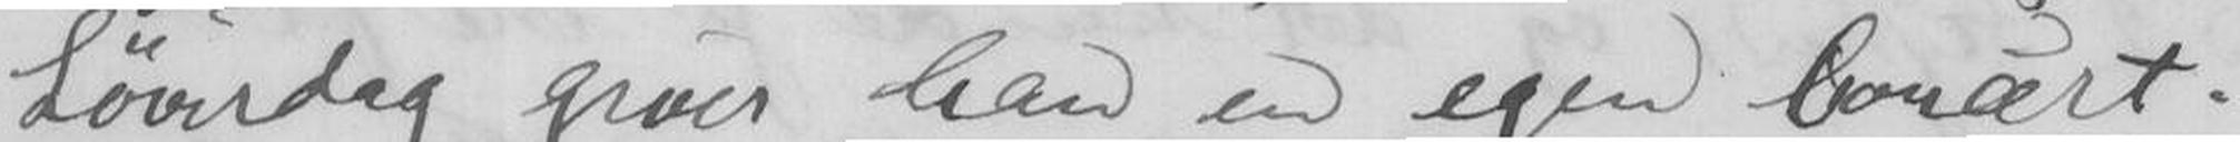Lørdag giver han en egen Concert.
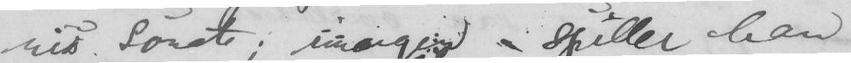nis Sonate; imorgen - spiller han
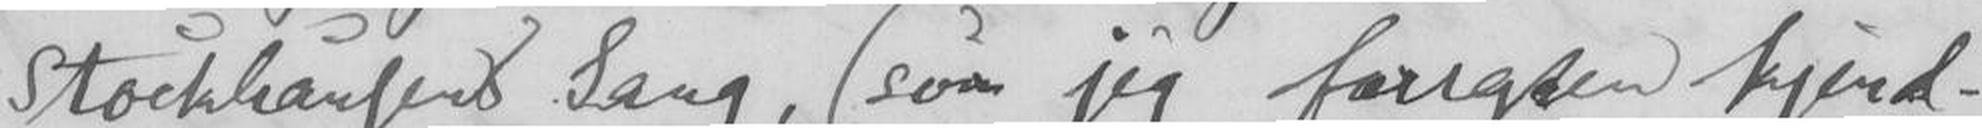Stockhansens Sang, (som jeg forresten kjend-
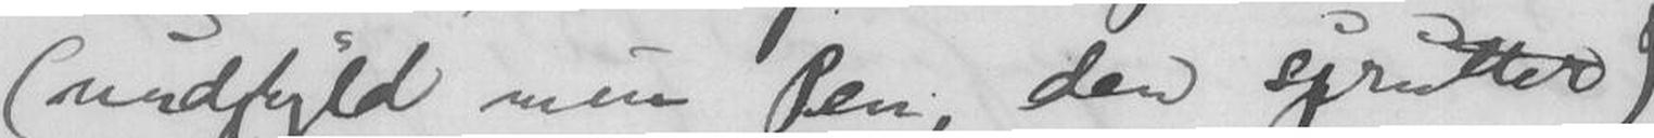(undskyld min Pen, den sprutter)
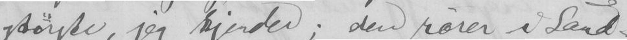største, jeg kjender; den rører i Sand
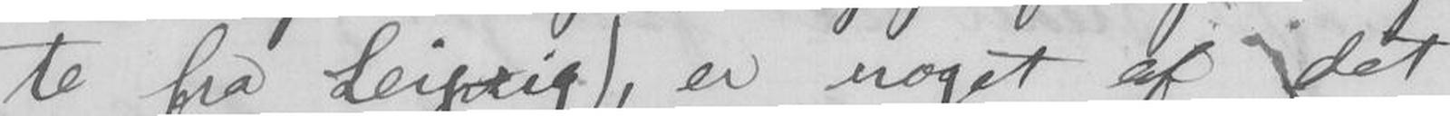te fra Leipzig), er noget af det
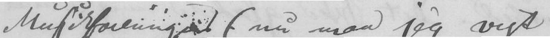Musikforeningens (nu maa jeg vist
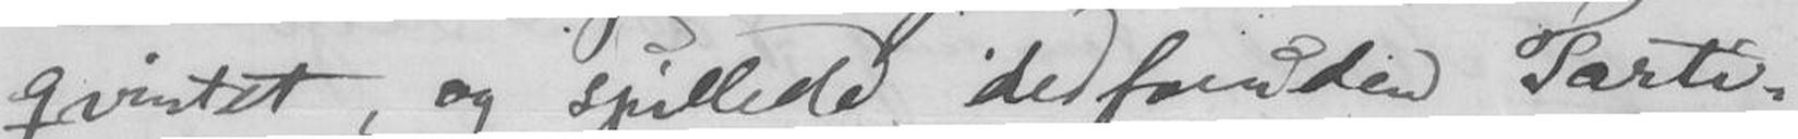qvintet, og spillede desforuden Tarti-
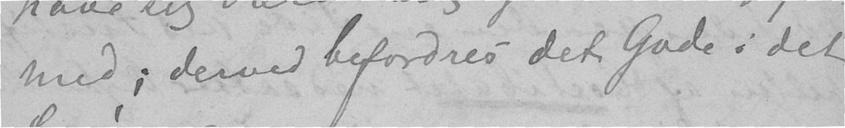med; derved befordres det Gode i det
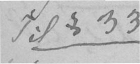Til § 33
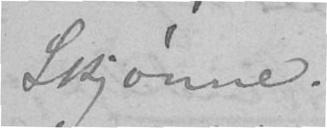Skjønne.
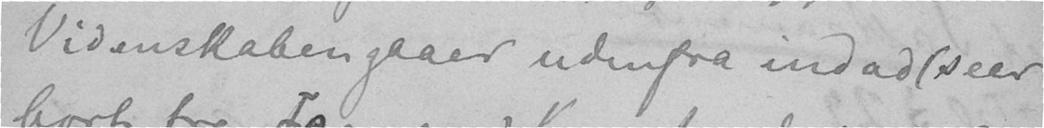Videnskaben gaaer udenfra indad (seer
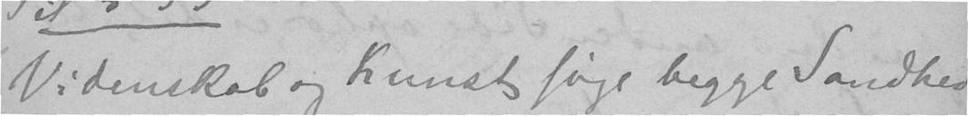Videnskab og Kunst søge begge Sandhed
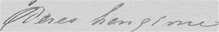Deres hengivne
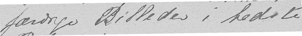færdige Billeder i bedste
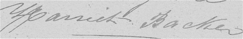Harriet Backer
